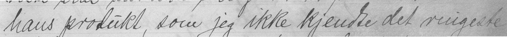hans produkt, som jeg ikke kjendte det ringeste
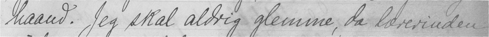haand. Jeg skal aldrig glemme, da lærerinden
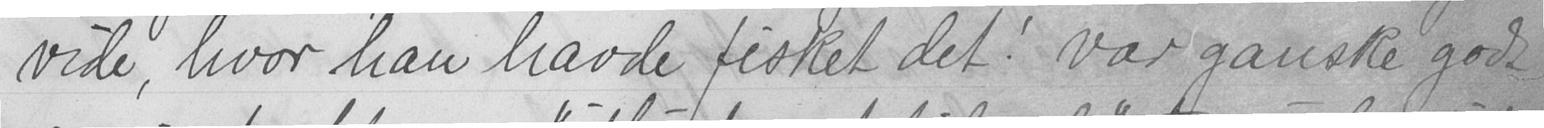vide, hvor han havde fisket det! var ganske godt
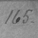165.
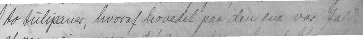to tulipaner, hvoraf hovedet paa den ene var faldt
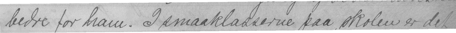bedre for ham. I smaaklasserne paa skolen er det
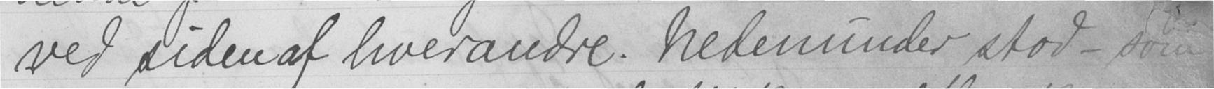ved siden af hverandre. Nedenunder stod - som
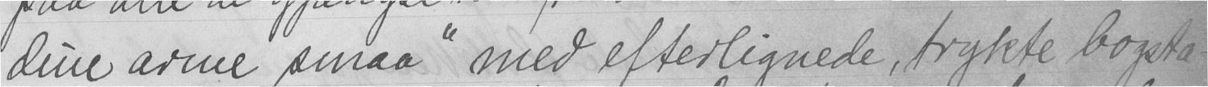dine arme smaa" med efterlignede, trykte bogsta
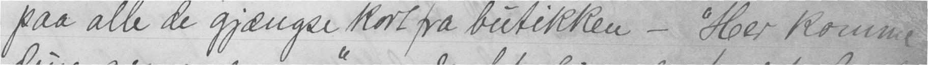paa alle de gjængse kort fra butikken - "Her komme
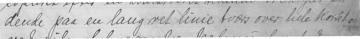dende paa en lang ret linie tværs over hele kortet og
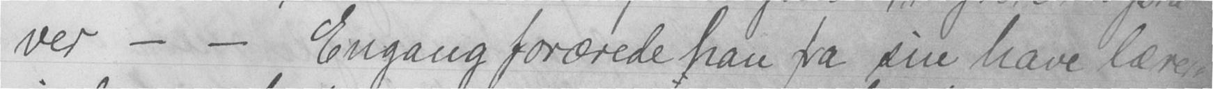ver - - Engang forærede han fra sin have lærer-
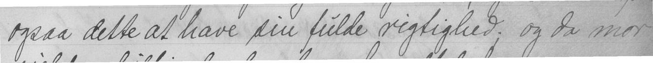ogsaa dette at have sin fulde rigtighed; og da mor
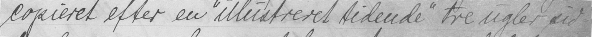copieret efter en "illustreret tidende" tre ugler sid-
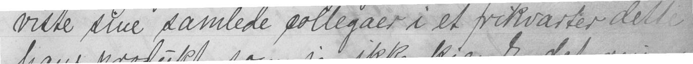viste sine samlede collegaer i et frikvarter dette
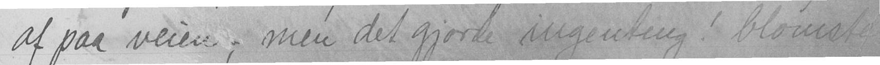af paa veien; men det gjorde ingenting! blomste
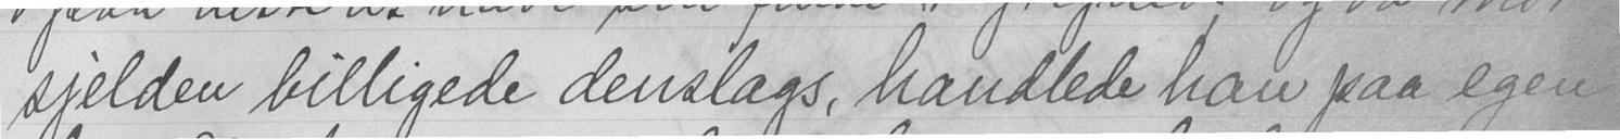sjælden billigede denslags, handlede han paa egen-
